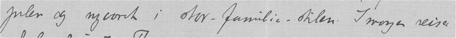julen og nyaaret i stor-familie-stilen. Imorgen reiser
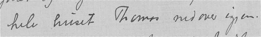hele huset Thomas nedover igjen.
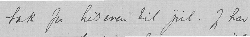tak for hilsenen til jul. Jeg har
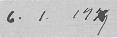6.1.1929
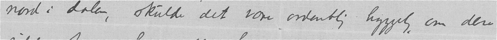nord i dalen, skulde det være ordentlig hyggelig om dere
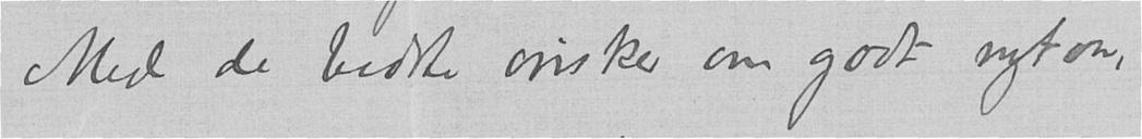Med de bedste ønsker om godt nytaar
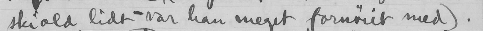skiold lidt - var han meget fornøiet med).
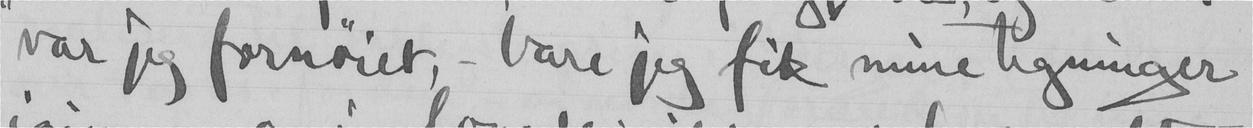var jeg fornøiet - bare jeg fik mine tegninger
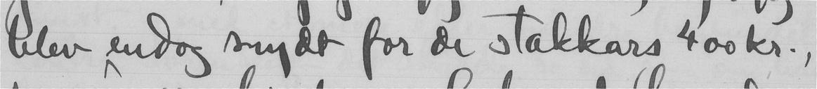blev endog snydt for de stakkars 400 kr.,
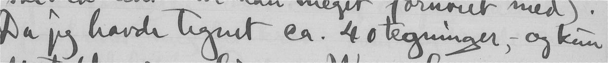Da jeg havde tegnet ca. 40 tegninger, - og kun
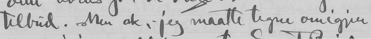tilbud. Men ak, - jeg maatte tegne omigjen
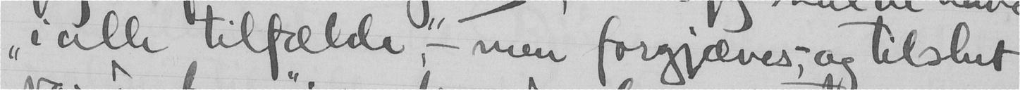"i aille tilfælde" - men forgjæves, og tilslut
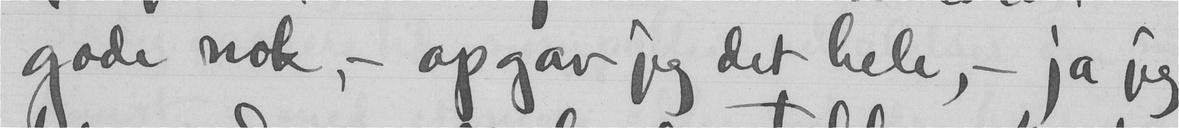gode nok, - opgav jeg det hele - ja jeg
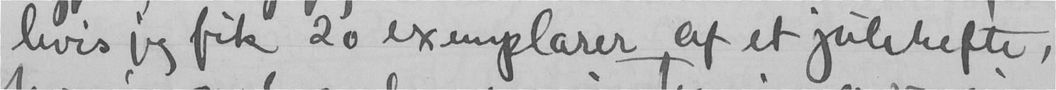hvis jeg fik 20 exemplarer af et julehefte,
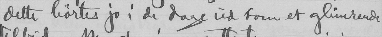dette hørtes jo i de dage ud som et glimrende
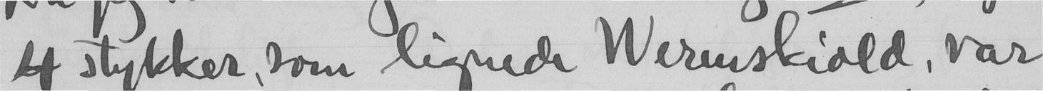4 stykker, som lignede Werenskiold, var
